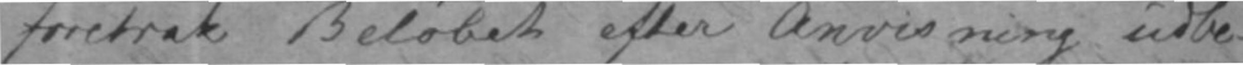foretrak Beløbet efter Anvisning udbe-
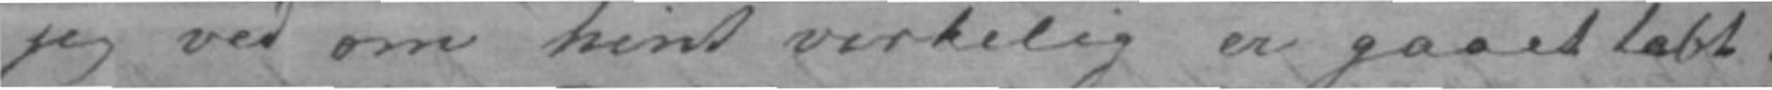jeg ved om hint virkelig er gaaet tabt.
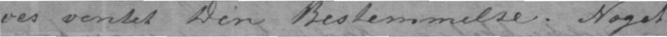ves ventet Din Bestemmelse. Noget
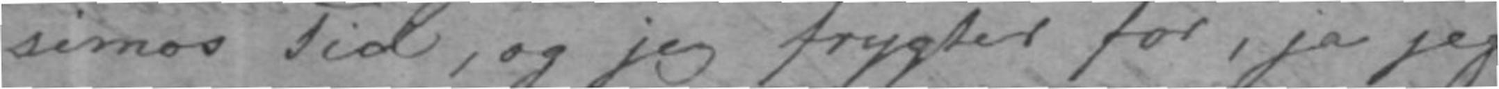simos Tid, og jeg frygter for, ja jeg
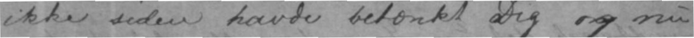ikke siden havde betænkt Dig ogom nu
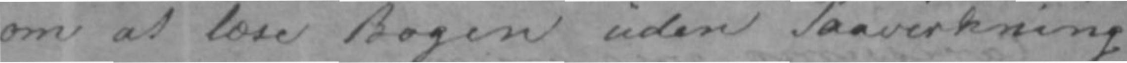om at læse Bogen uden Paavirkning
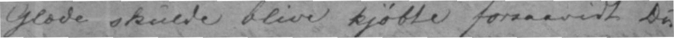Glæde skulde blive kjøbte forsaavidt Du
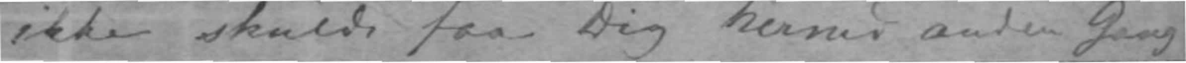ikke skulde faa Dig herned anden Gang.
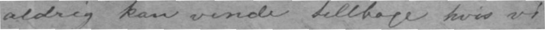aldrig kan vende tillbage hvis vi
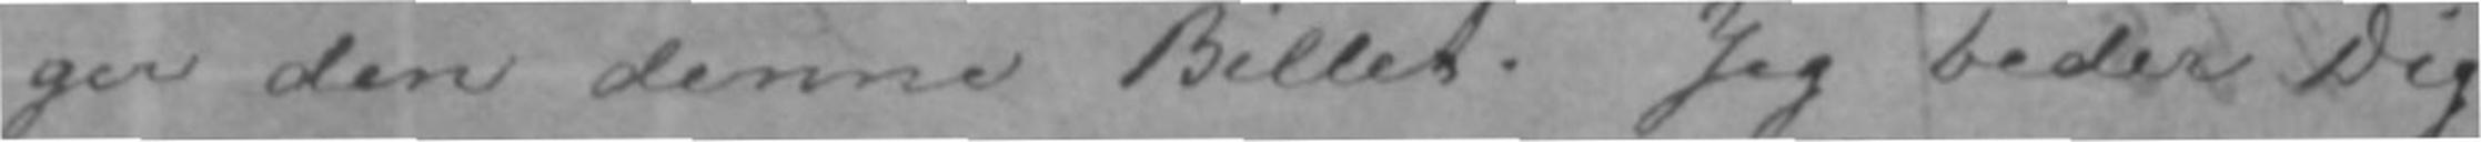ger den denne Billet. Jeg beder Dig
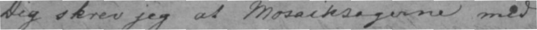Dig skrev jeg at Mosaiksagerne med
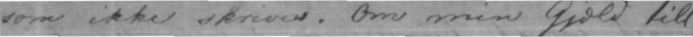som ikke skrives. Om min Gjæld till
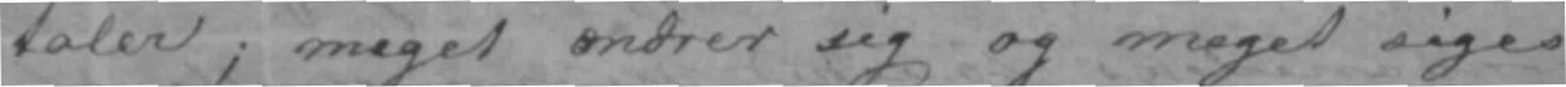taler; meget ændrer sig og meget siges
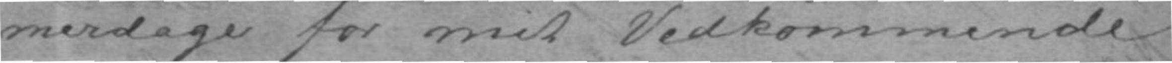merdage for mit Vedkommende
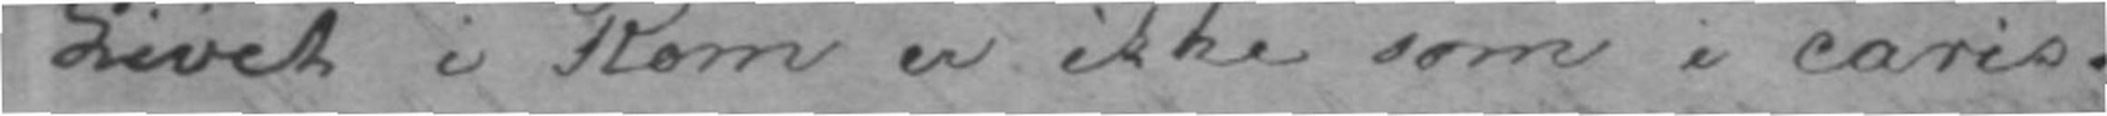Livet i Rom er ikke som i caris-
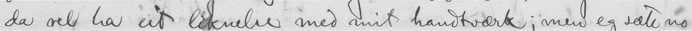da vel ha eit liknelse med mit handtværk; men eg sæte no
